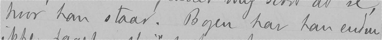hvor han staar. Bogen har han endnu
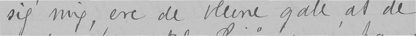sig mig, ere de blevne gale, at de
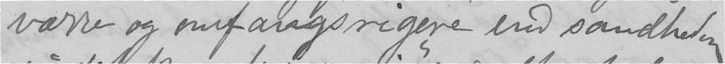værre og omfangsrigere end sandheden
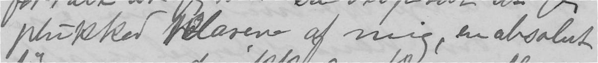plukked klærne af mig, en absolut
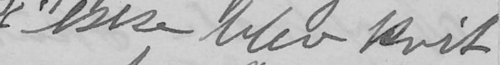ikke blev kvit
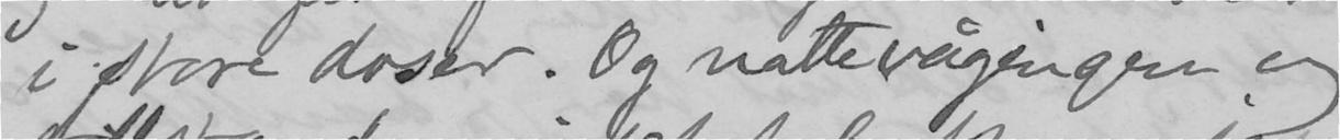i store doser. Og nattevågingen og
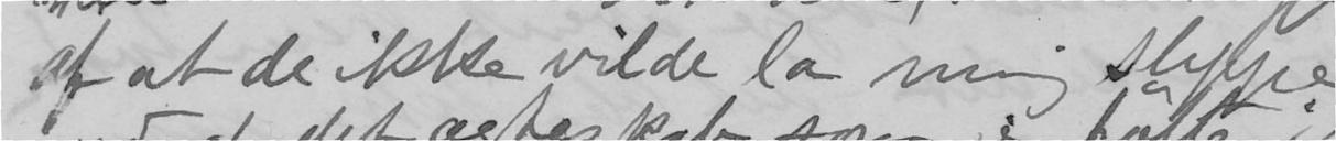af at de ikke vilde la mig slippe
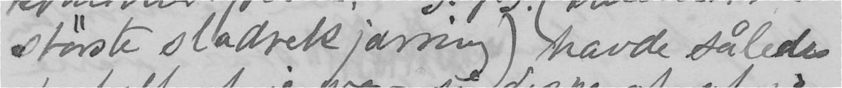største sladrekjærring) havde således
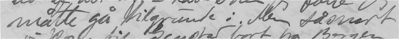måtte gå tilgrunde i. Men såsnart
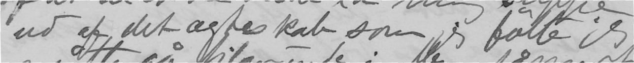ud af det ægteskab som jeg følte jeg
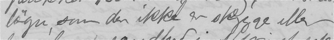løgn, som der ikke er skygge eller
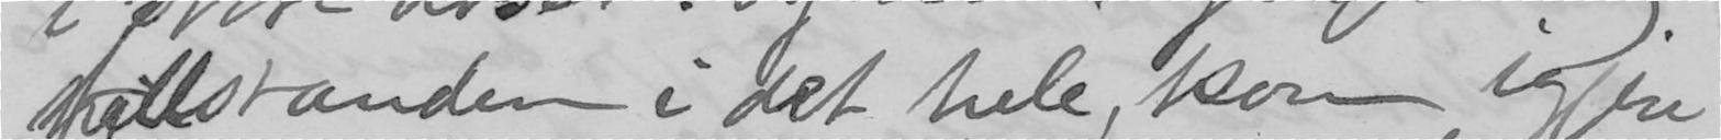tillstanden i det hele, kom igjen
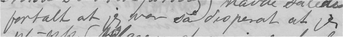fortalt at jeg var så desperat at jeg
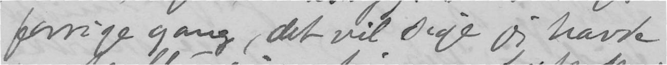forrige gang, det vil sige jeg havde
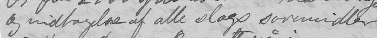og indtagelse af alle slags sovemidler
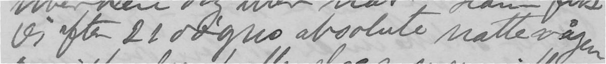jeg eften 21 dagers absolute nattevågen
